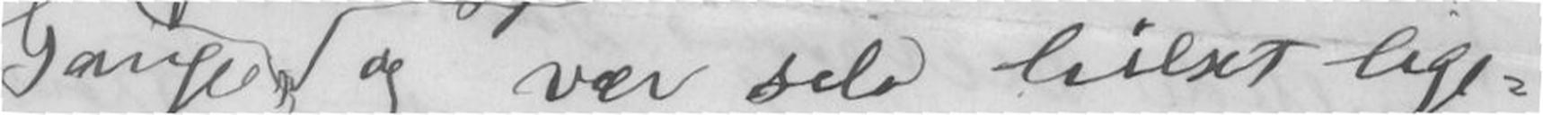Gange og vær selv hilset lige-
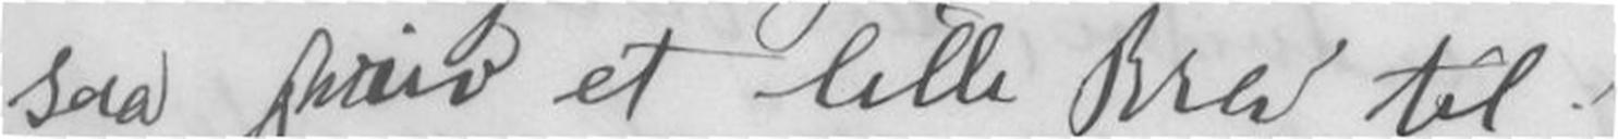saa skriv et lille Brev til!
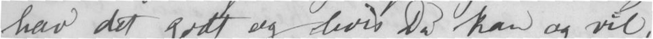hav det godt og hvis Du kan og vil,
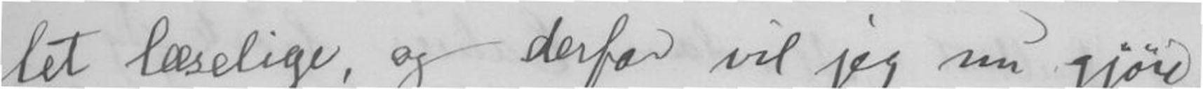let læselige, og derfor vil jeg nu gjøre
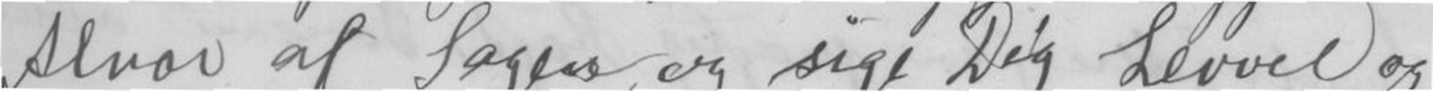Alvor af Sagen og sige Dig Levvel og
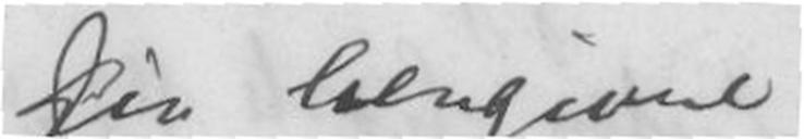Din hengivne
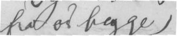fra os begge
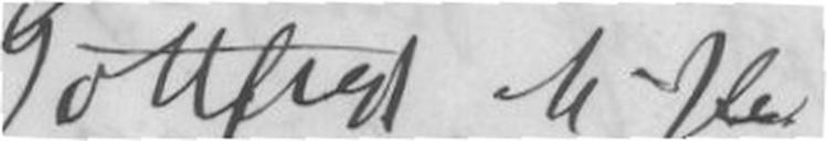Gottfred M-H
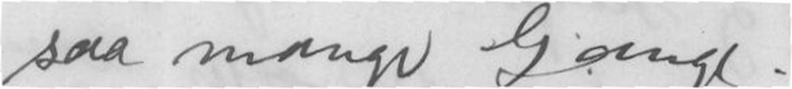saa mange Gange.
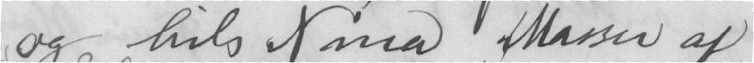og hils Nina Masser af
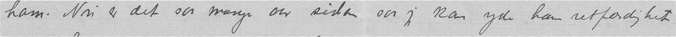ham. Nu er det saa mange aar siden saa jeg kan yde ham retfærdighet
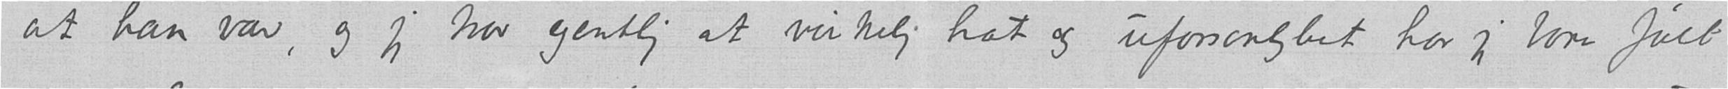at han var, og jeg tror egentlig at virkelig hat og uforsonlighet har jeg bare følt
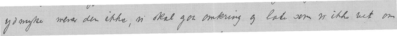ydmyke mener den ikke, vi skal gaa omkring og late som vi ikke vet om
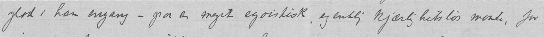glad i ham engang – paa en meget egoistisk, egentlig kjærlighetsløs maate, for
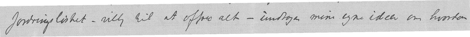fordringsløshet – villig til at offre alt – undtagen mine egne ideer om hvordan
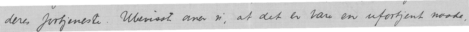deres fortjeneste. Ubevisst aner vi, at det er bare en ufortjent naade,
